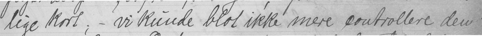lige kort; - vi kunde blot ikke mere controllere dem-
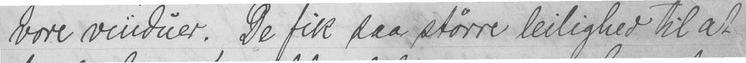vore vinduer. De fik saa større leilighed til at
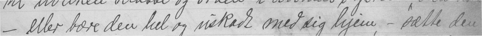- eller bære den hel og uskadt med sig hjem, - sætte den
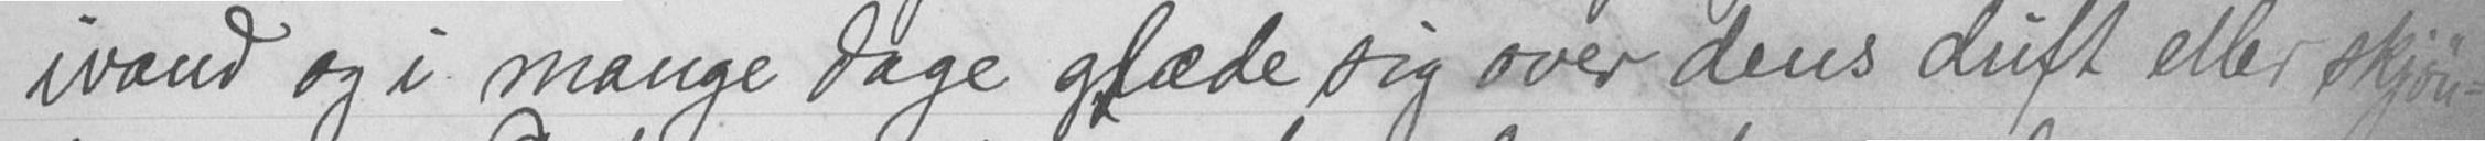ivand og i mange dage glæde sig over dens duft eller skjøn-
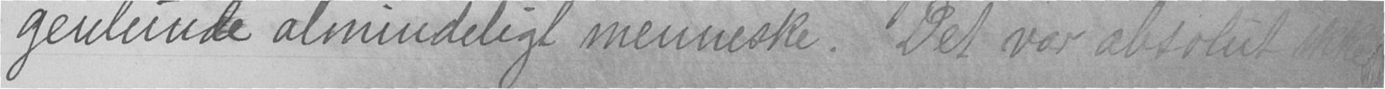genlunde almindeligt menneske. Det var absolut ikke
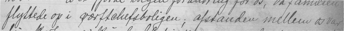flyttede op i værftchefsboligen; afstanden mellem os var
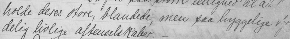holde deres store, blandede, men saa hyggelige
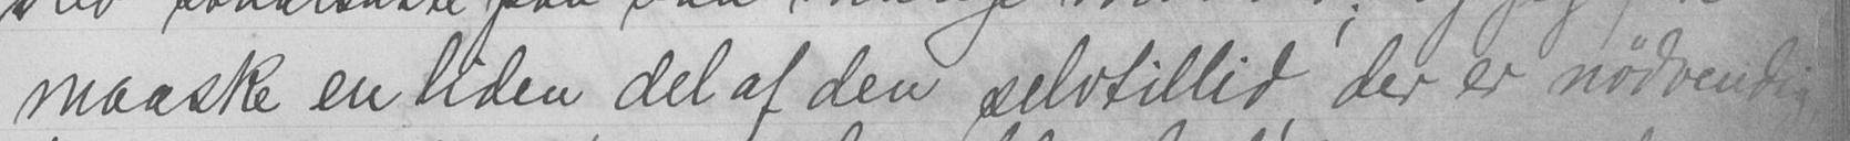maaske en liden del af den selvtillid, der er nødvendig,
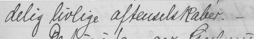delig livlige aftenselskaber. -
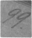99.
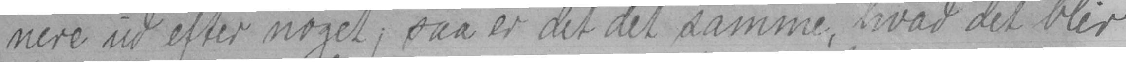nere ud efter noget; saa er det det samme, hvad det blir
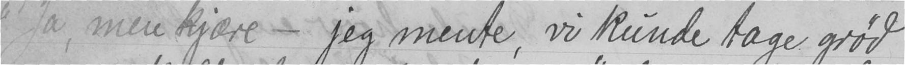"Ja, men kjære - jeg mente, vi kunde tage grød
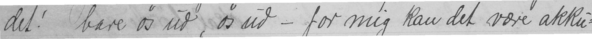det! bare os ud, os ud - for mig kan det være akku-
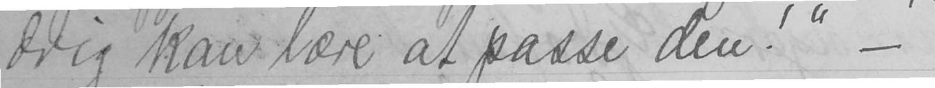drig kan lære at passe den!" -
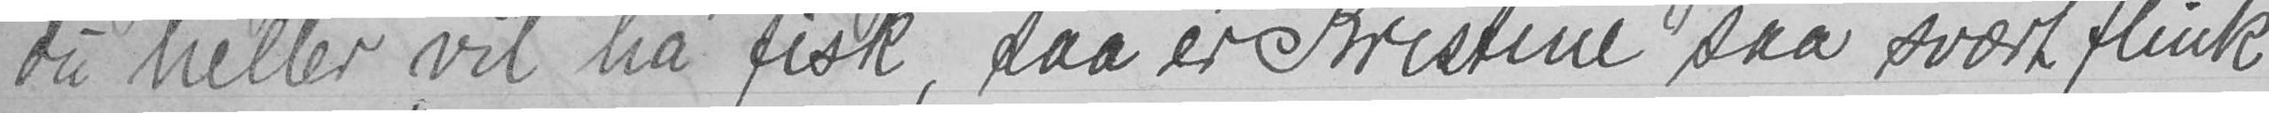du heller vil ha fisk, saa er Kristine saa svært flink
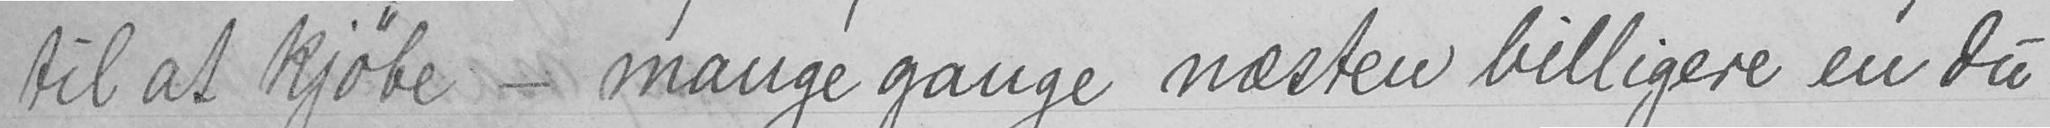til at kjøbe - mange gange næsten billigere en du
